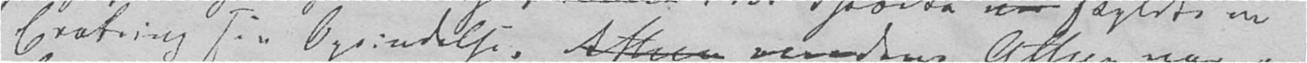Erobring sin Oprindelse, Athen medens Athen var op-
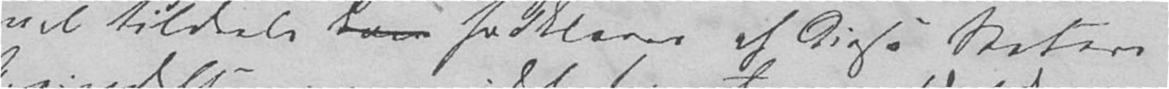vel tildeels kan forklares af disse Staters
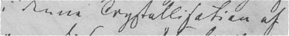i denne Crystallitation af
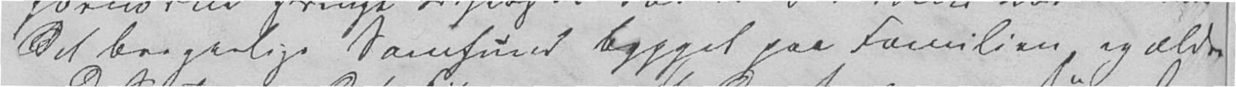det borgerlige Samfund bygget paa Familien og ældre
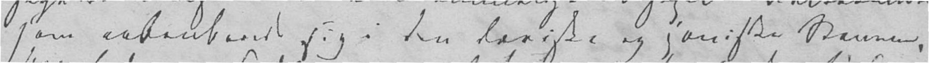som aabenbarede sig i den doriske og joniske Stamme,
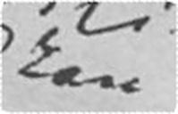kan
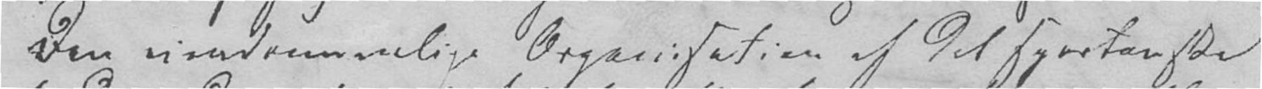Den eiendommelige Organisation af det spartanske
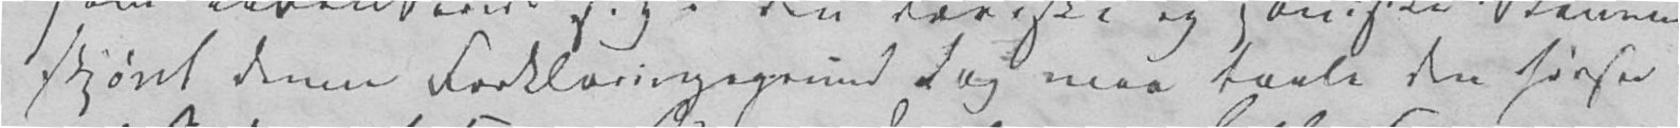skjønt denne Forklaringsgrund dog maa taale den første
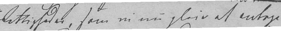Rettigheder, som vi nu pleier at antage
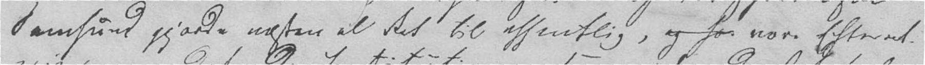Samfund gjorde næsten al Ret til offentlig, og har vore Efterret-
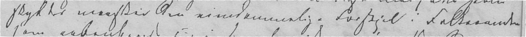skyldes maaskee den eiendommelige Forskjel i Folkeaanden
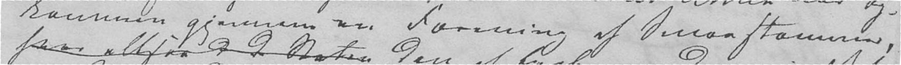kommen gjennem en Forening af Smaastammer,
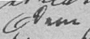dem
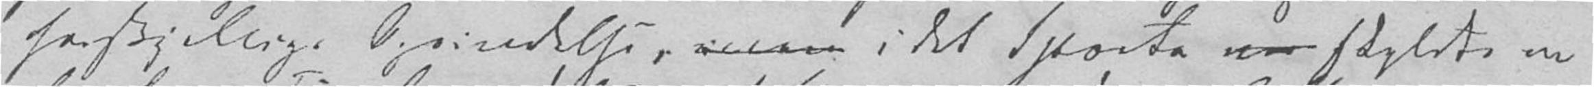forskjellige Oprindelse, eller i det Sparta var skyldte en
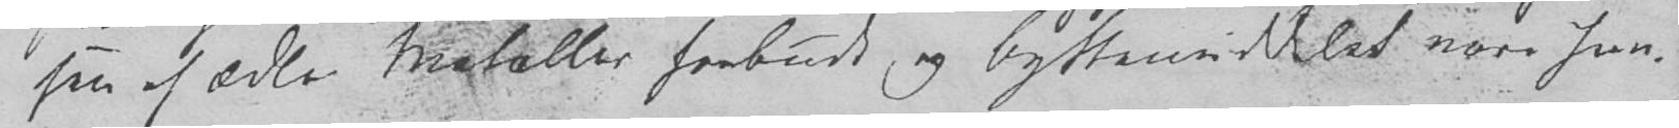sen af ædle Metaller forbudt, og Byttemiddelet var Jern-
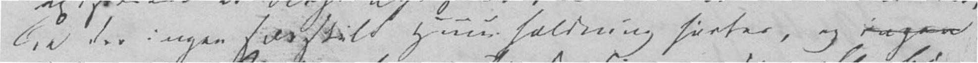da der ingen særskilt Huusholdning førtes, og ingen
